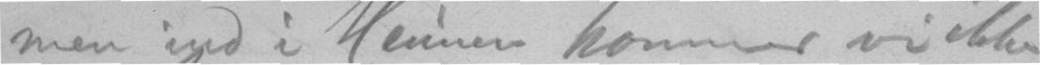men ind i Heimen kommer vi ikke,
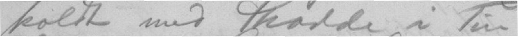koldt med Skodde i Tin-
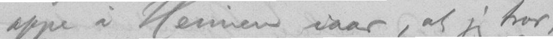oppe i Heimen iaar, at jeg tror,
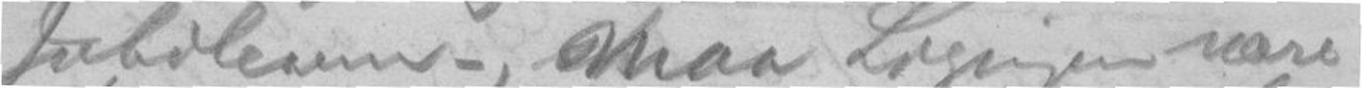Jubileum -, maa Ligningen være
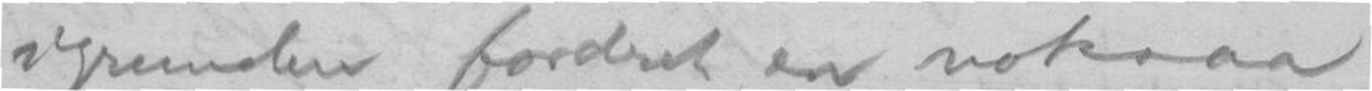igrunden fordret en noksaa
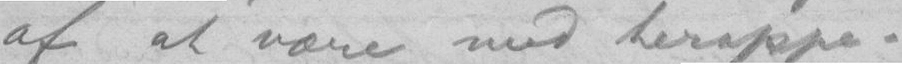af at være med heroppe.
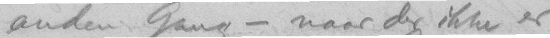anden Gang - naar der ikke er
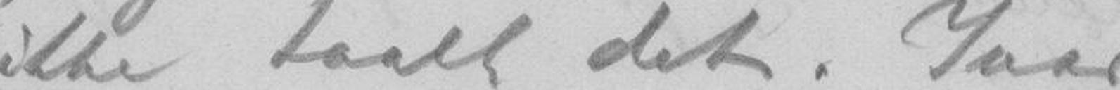jeg ikke taalt det. Iaar
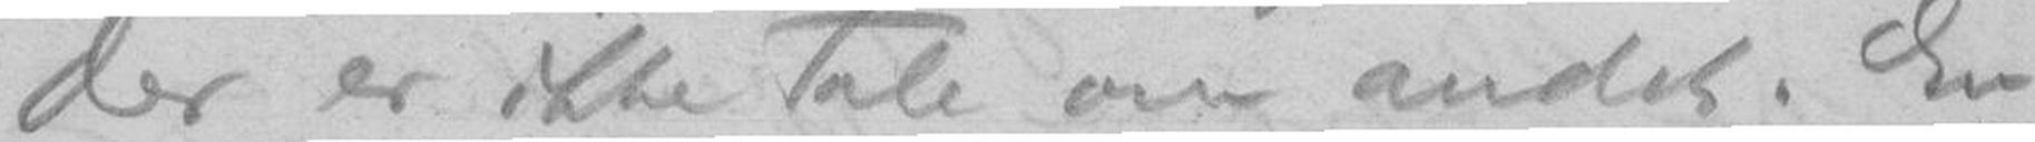der er ikke Tale om andet. En
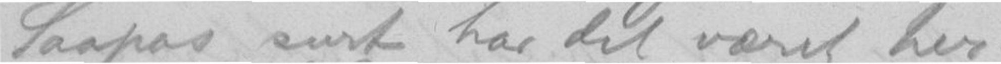Saapas surt har det været her-
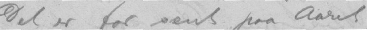Det er for sent paa Aaret,
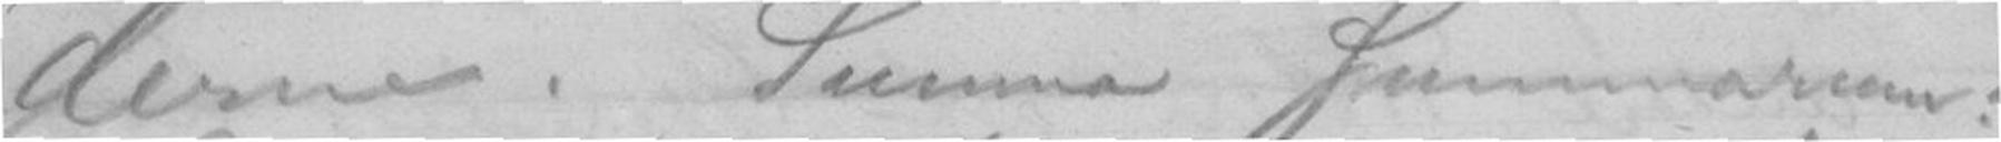derne. Summa Summarum:
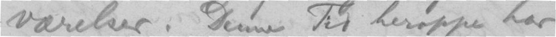værelser. Denne Tid heroppe har
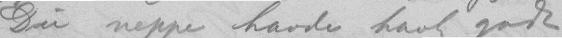Du neppe havde havt godt
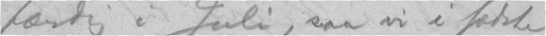færdig i Juli, saa vi i sidste
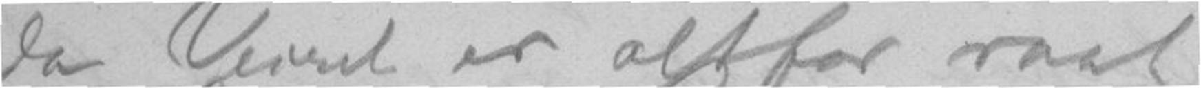da Veiret er altfor vaat og
